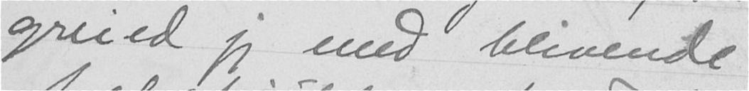greied jeg med blivende
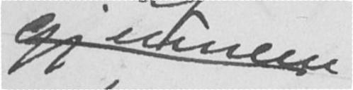gjennem
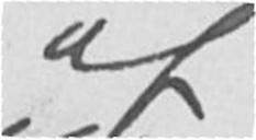af
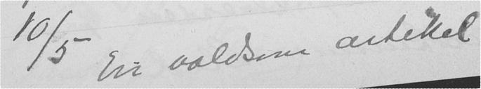10/5 En voldsom artikel
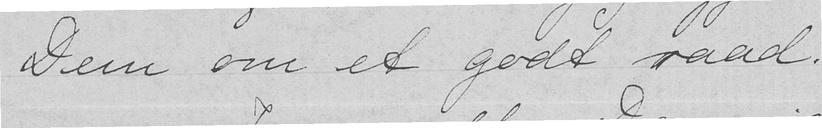Dem om et godt raad.
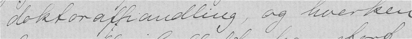doktorafhandling, og hverken
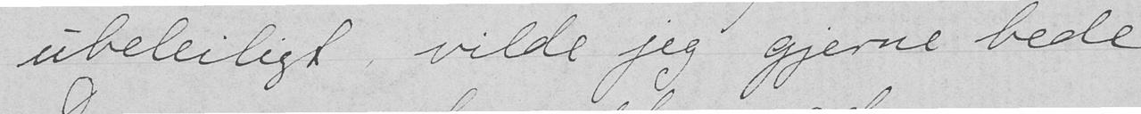ubeleiligt, vilde jeg gjerne bede
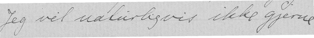Jeg vil naturligvis ikke gjerne
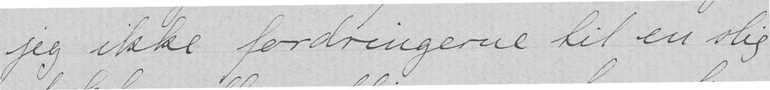jeg ikke fordringerne til en slig
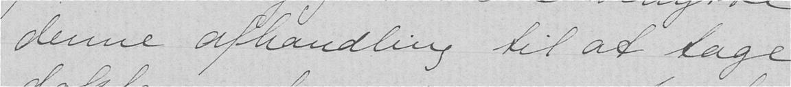denne afhandling til at tage
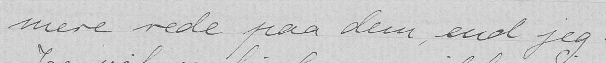mere rede paa dem, end jeg.
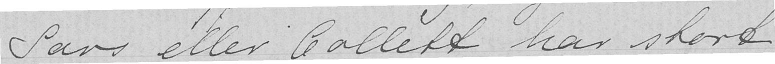Sars eller Collett har stort
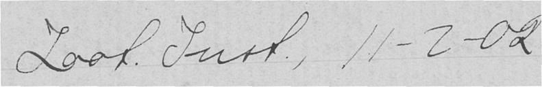Zoot. Inst., 11-2-02
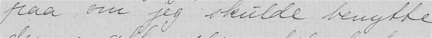paa, om jeg skulde benytte
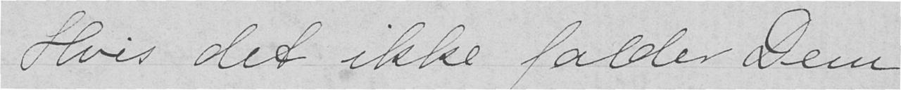Hvis det ikke falder Dem
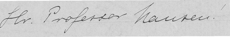Hr. Professor Nansen!
